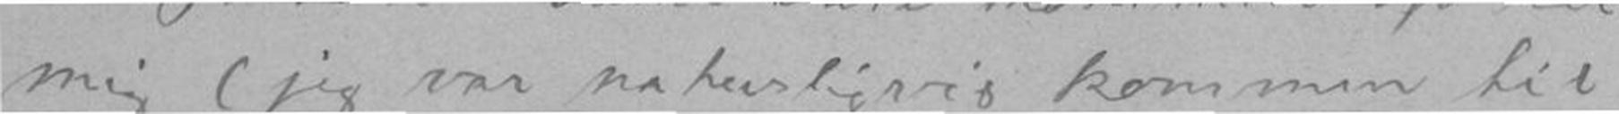mig (jeg var naturligvis kommen til
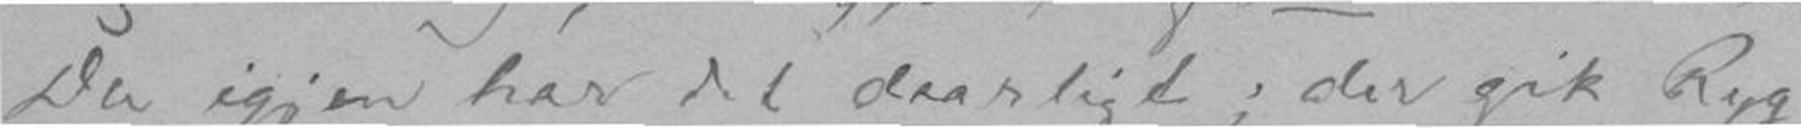Du igjen har det daarligt; der gik Ryg-
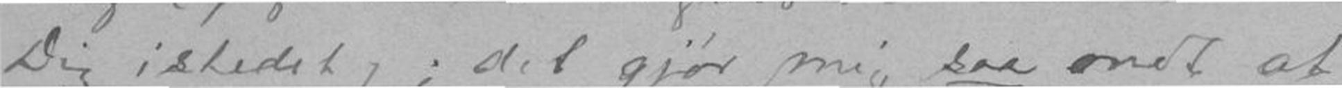Dig istedet), det gjør mig saa ondt at
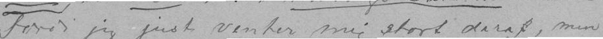fordi jeg just venter mig stort deraf, men
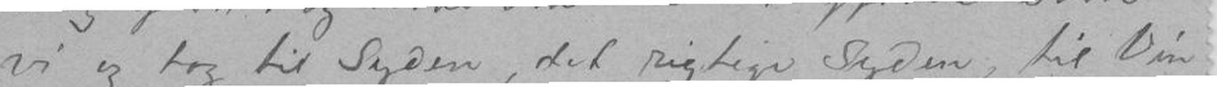vi og tog til Syden, det rigtige Syden, til Vin-
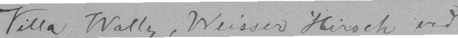Villa Wally, Weisser Hirsch ved
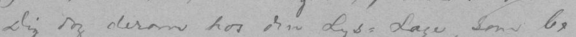Dig dog derom hos den Lys= Lage, som be-
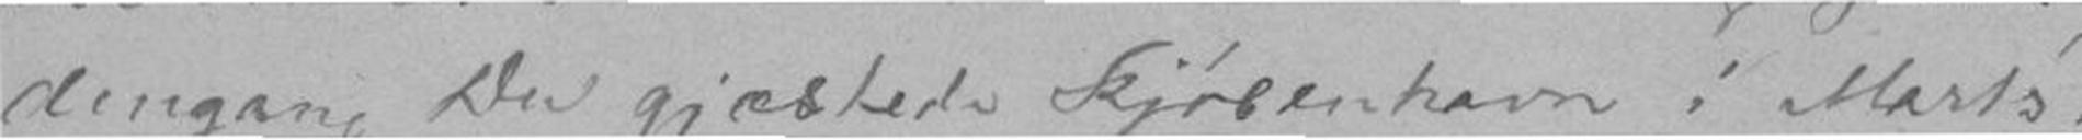dengang Du gjæstede Kjøbenhavn i Marts.
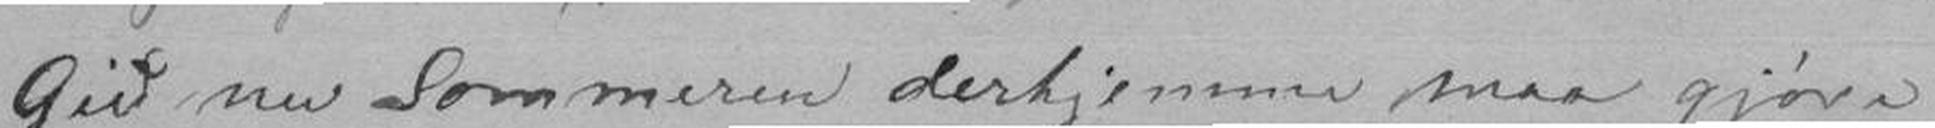Gid nu Sommeren derhjemme maa gjøre
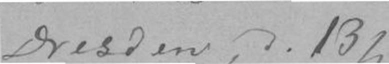Dresden, d. 13/5
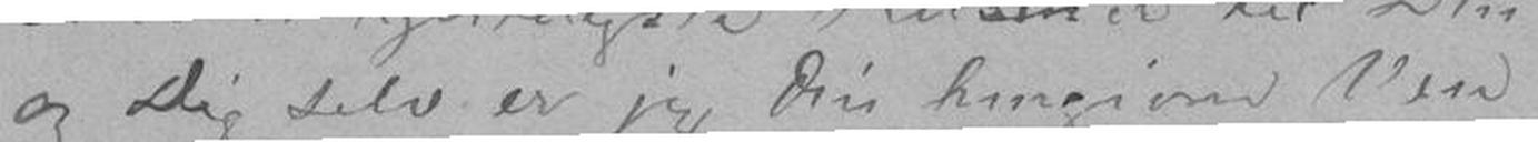og Dig selv er jeg Din Hengivne Ven.
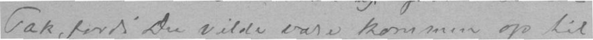Tak fordi Du vilde være kommen op til
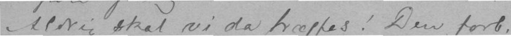Aldrig skal vi da træffes! Den forb.
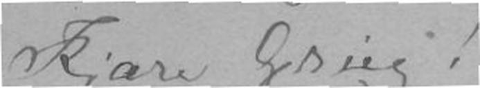Kjære Grieg!
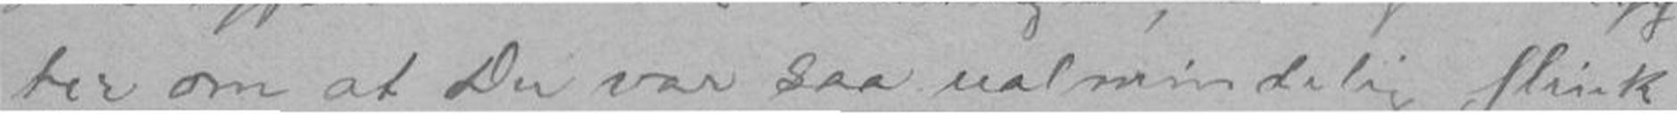ter om at Du var saa ualmindelig flink,
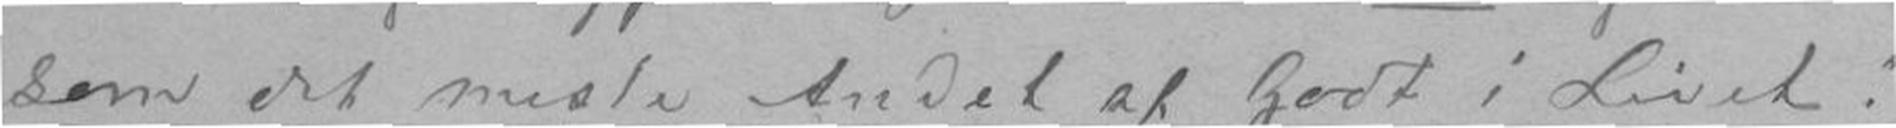som det meste andet af Godt i Livet!
